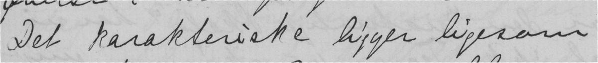Det karakteriske ligger ligesom
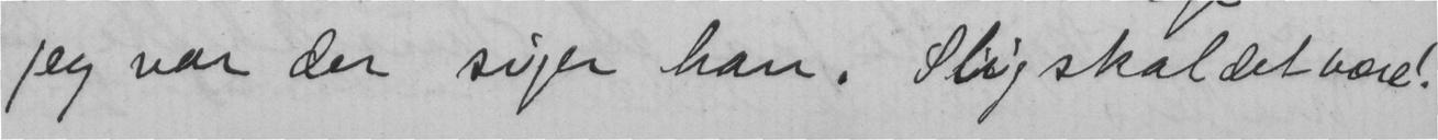Jeg var der siger han. Slig skal det være!
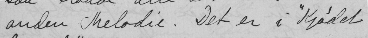anden melodie. Det er i "Kjødet
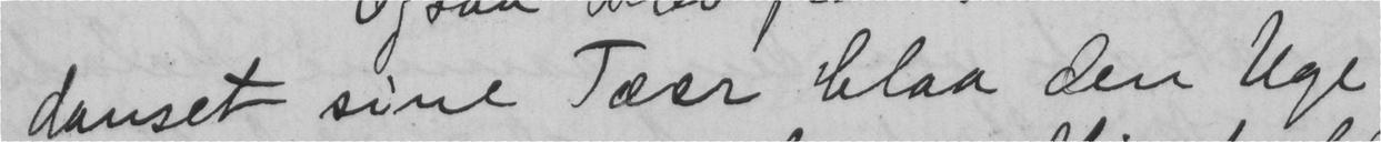danset sine Tæer blaa den Uge
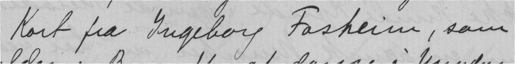Kort fra Ingeborg Fosheim, som
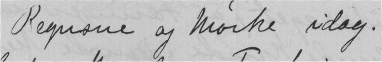Regnsne og Mørke idag.
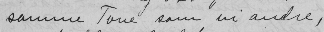samme Tone som vi andre,
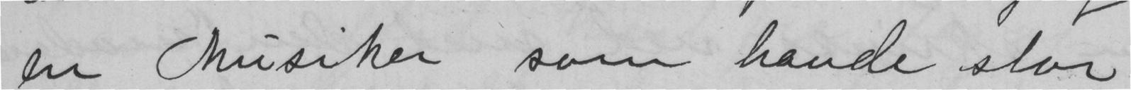en Musiker som havde stor
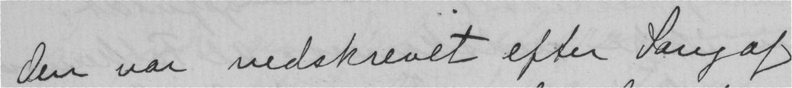den var nedskrevet efter Sang af
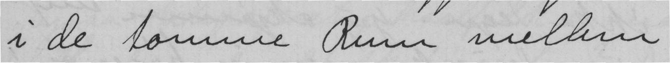i de tomme Rum mellem
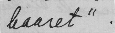baaret".
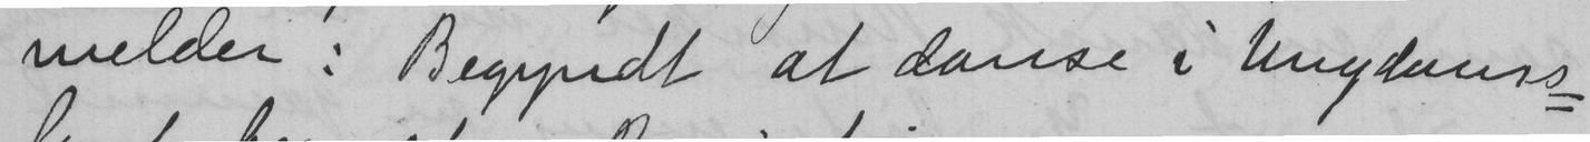melder: Begyndt at danse i Ungdoms-
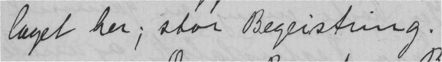laget her; stor Begeistring.
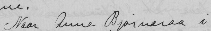Naar Anne Bjørnaraa i
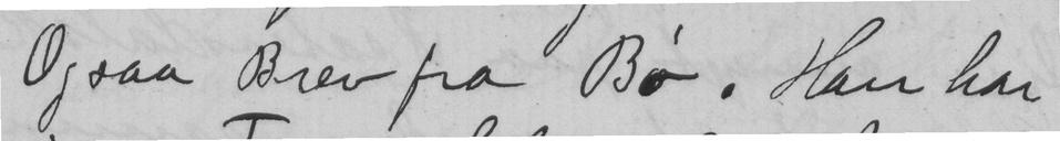Ogsaa Brev fra Bø. Han har
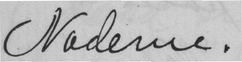Noderne.
